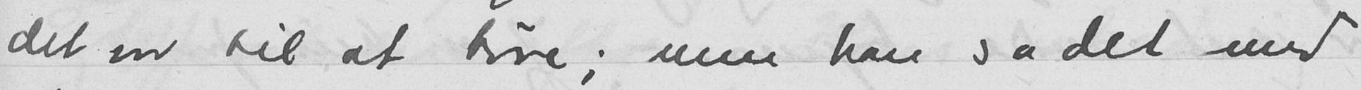det var til at høre; men han sa det med
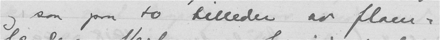og saa paa to billeder av flam-
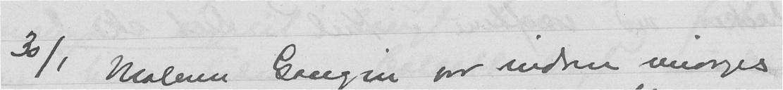30/1 Maleren Gaugin var indom imorges
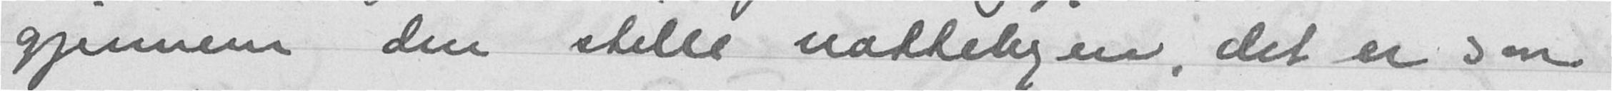gjennem den stille nattelyen, det er saa
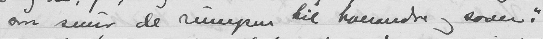saa snur de rumpen til hverandre og sover."
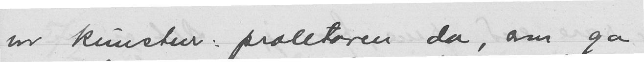var kunstner-proletaren da, som ga
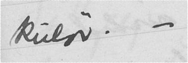kulør. -
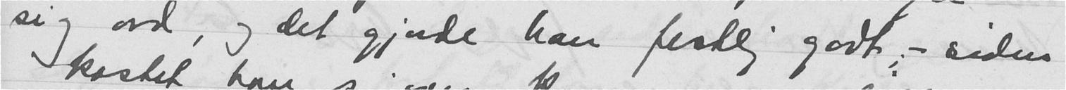sig ord, og det gjorde han festlig godt; - siden
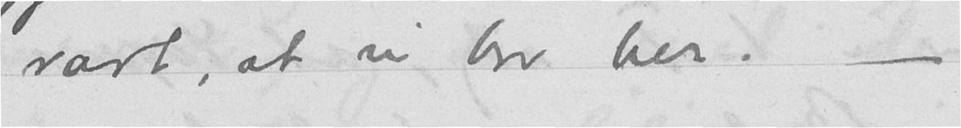rart, at vi bor her. -
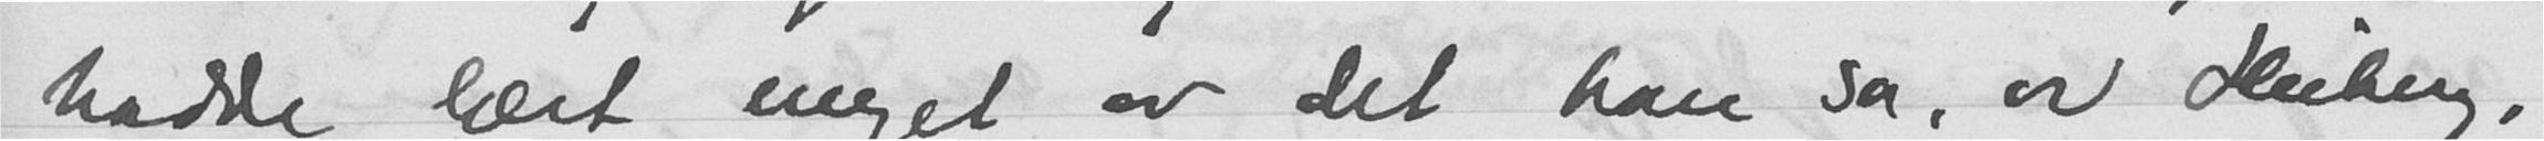hadde lært meget av det han sa, av Heiberg,
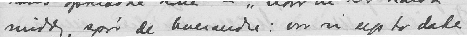middag, spør de hverandre: var vi up to date
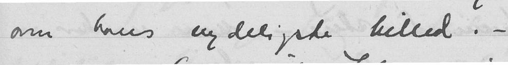som hans nydeligste billed. -
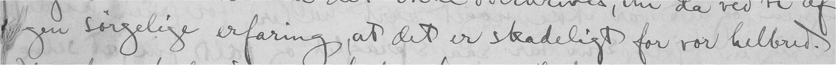egen sørgelige erfaring, at det er skadeligt for vor helbred.
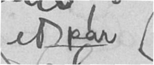et par
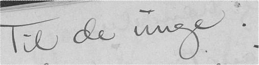Til de unge.
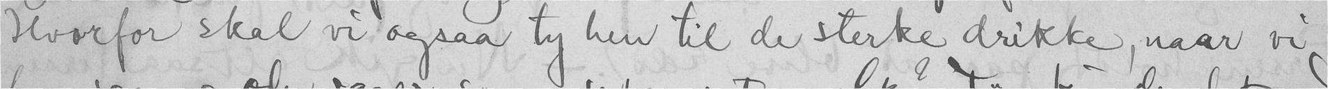Hvorfor skal vi ogsaa ty hen til de sterke drikke, naar vi
